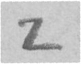2
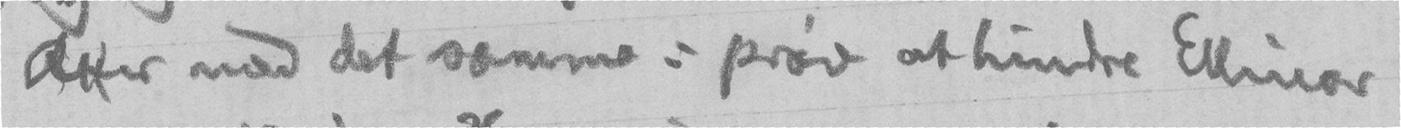Atter med det samme: prøv at hindre Ellinor
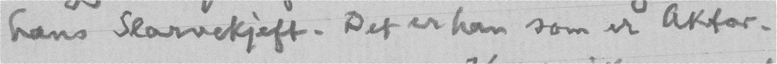hans Slarvekjeft. Det er han som er Aktor.
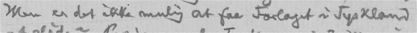Men er det ikke mulig at faa Forlaget i Tyskland
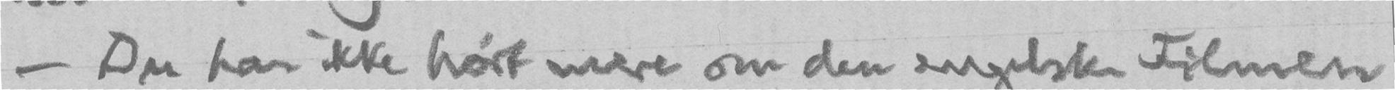- Du har ikke hørt mere om den engelske Filmen
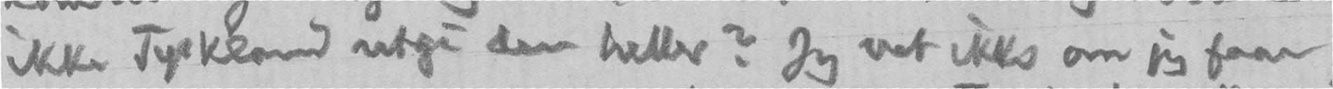ikke Tyskland utgi den heller? Jeg vet ikke om jeg faar
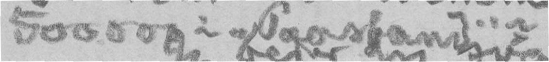500.000 i "Paastand"?
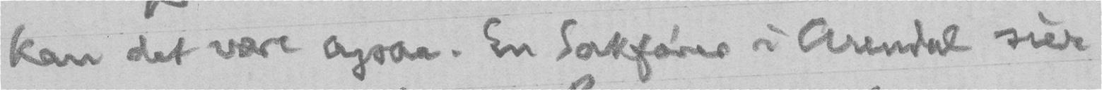kan det være ogsaa. En Sakfører i Arendal sier
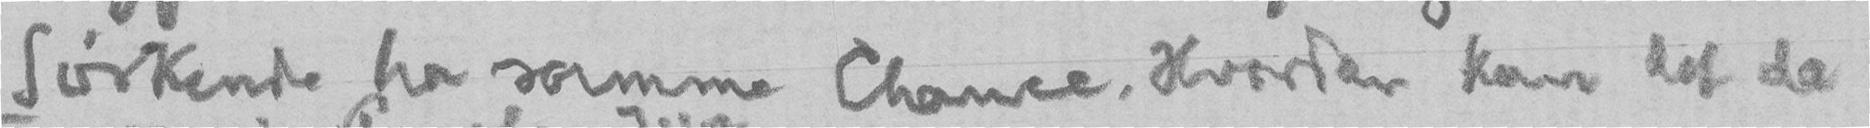Søskende ha samme Chance. Hvordan kan det da
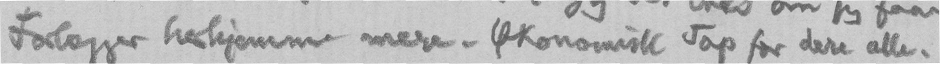Forlægger herhjemme mere. Økonomisk Tap for dere alle.
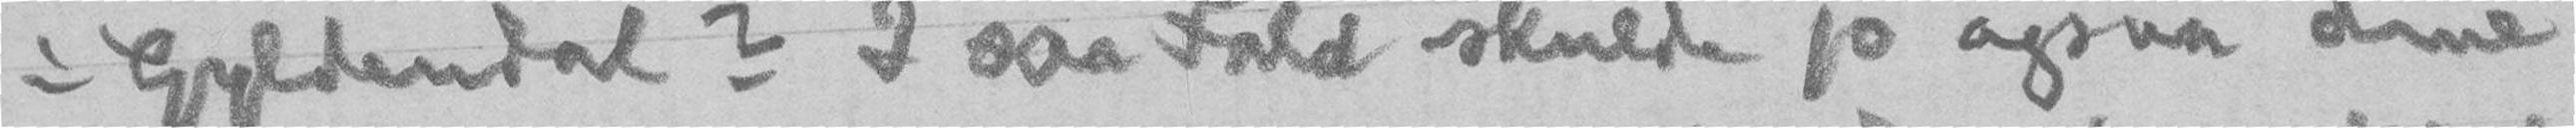i Gyldendal? I saa Fald skulde jo ogsaa dine
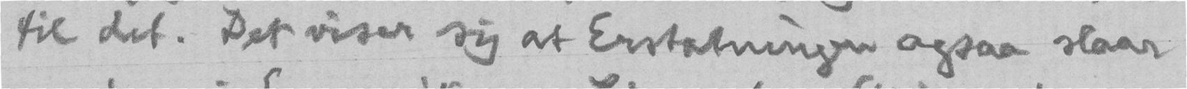til det. Det viser sig at Erstatningen ogsaa slaar
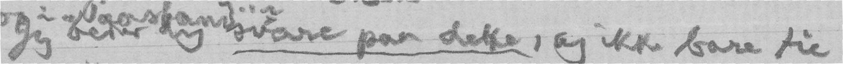Jeg beder dig svare paa dette, og ikke bare tie
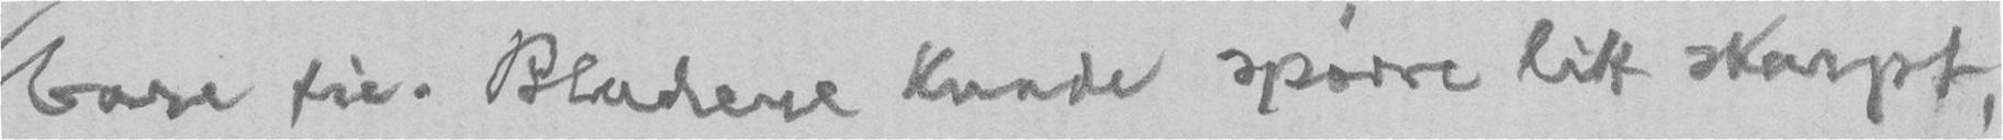bare tie. Bladene kunde spørre litt skarpt,
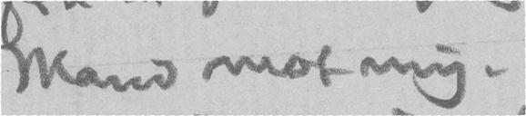Mand mot mig.
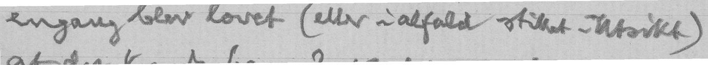engang blev lovet (eller ialfald stillet i Utsikt)
